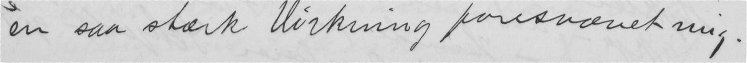en saa stærk Virkning foresvævet mig.
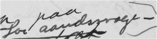for aandssvage -
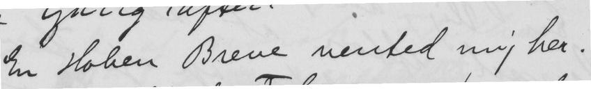En Hoben Breve vented mig her.
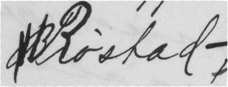Røstad -
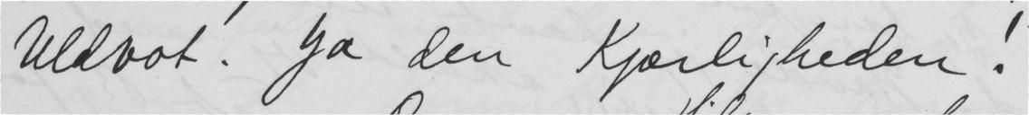Uldvot. Ja den Kjærligheden!
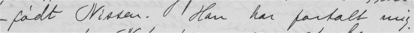- født Nissen. Han har fortalt mig
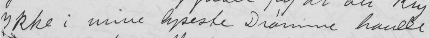Ikke i mine lyseste Drømme havde
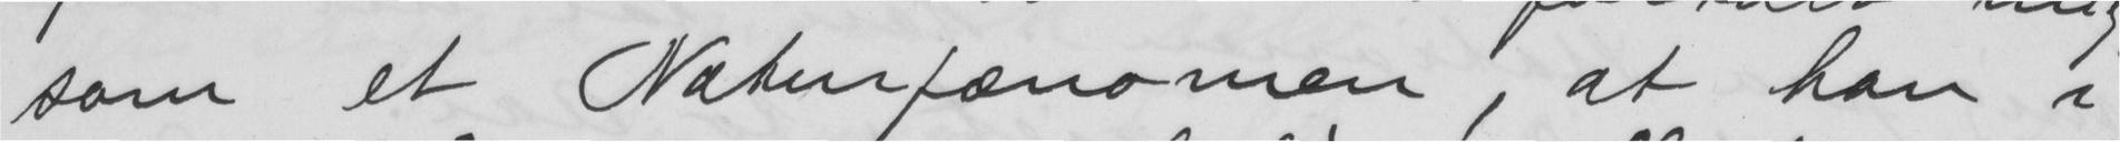som et Naturfænomen, at han i
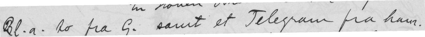Bl.a. to fra G. samt et Telegram fra ham.
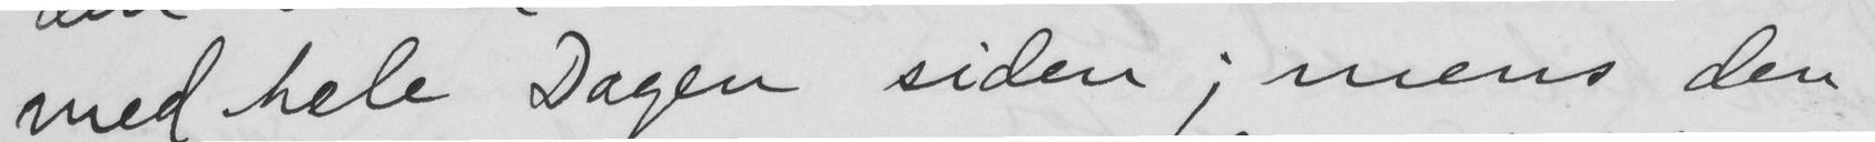med hele Dagen siden; mens den
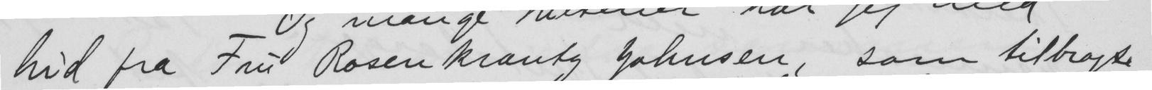hid fra Fru Rosenkrantz Johnsen, som tilbragte
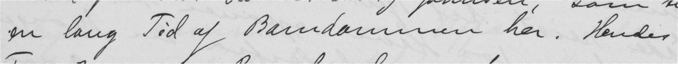en lang Tid af Barndommen her. Hendes
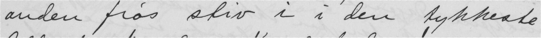anden fros sliv i i den tykkeste
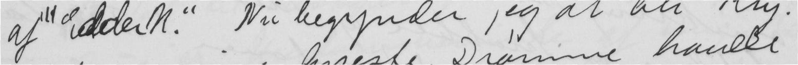af "Edderk." Nu begynder jeg at bli Kry.
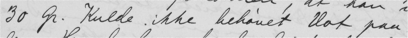30 Gr. Kulde ikke behøvet Vot paa
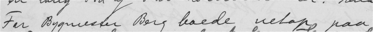Far Bygmester Berg boede netop paa
